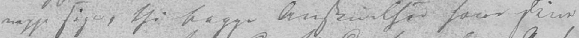neppe sige, thi begge Anskuelser have sine
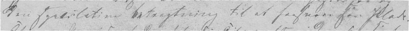den speculative Betragtning til at forsvare sin Plads.
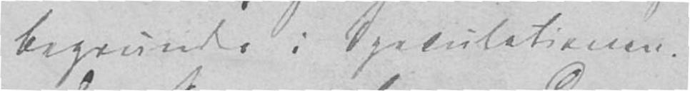begrundes i Speculationen.
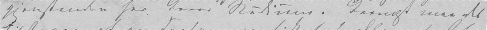gjenstanden for Deres Studium. Dernæst maa det
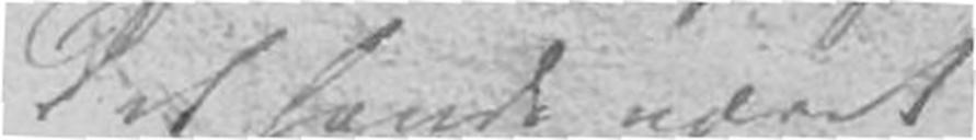Det havde været
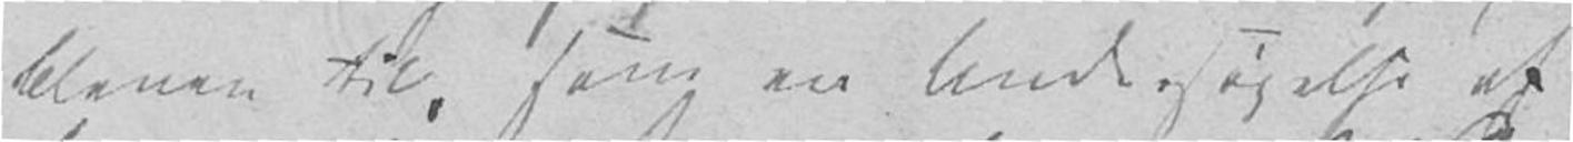bleven til, som en Undersøgelse af
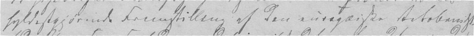fyldestgjørende Fremstilling af den europæiske Retsbevidst-
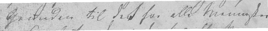Grunden til det for alle Mennesker
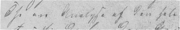Thi en Analyse af den hele
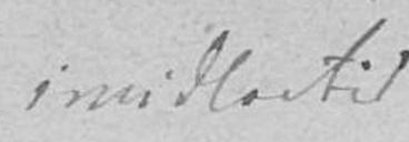imidlertid
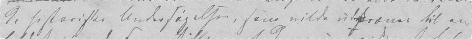de historiske Undersøgelser, som vilde udkræves til en
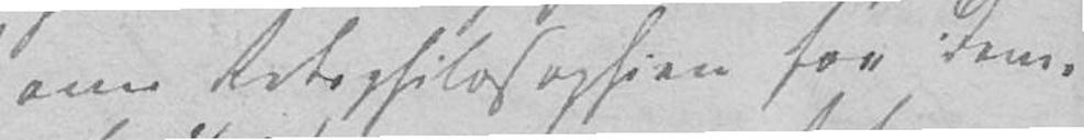om Retsphilosophien for Dem,
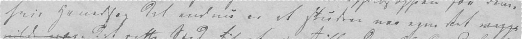hvis Hovedsag det endnu er at studere vor egen Ret neppe
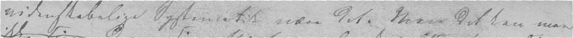videnskabelige Systematik være det. Men det kan man
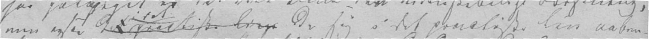men ogsaa de sig i det practiske Livs aaben-
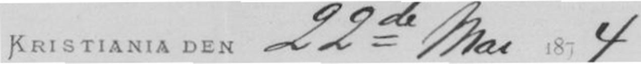KRISTIANIA DEN 22de Mai 1874
